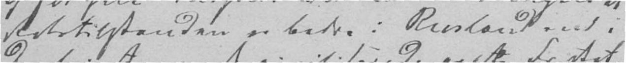Retstilstanden er bedre i Rusland end i
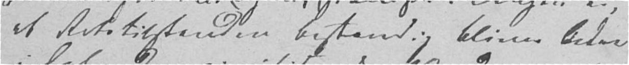at Retstilstanden bestandig bliver bedre
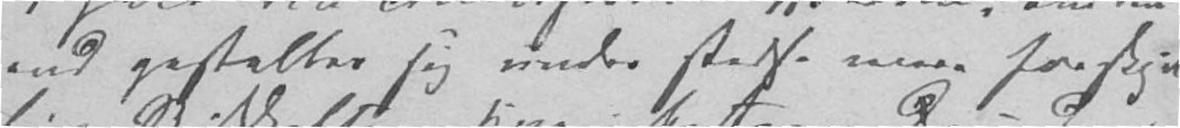end gestalter sig under stedse mere forskjel-
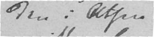den i Athen
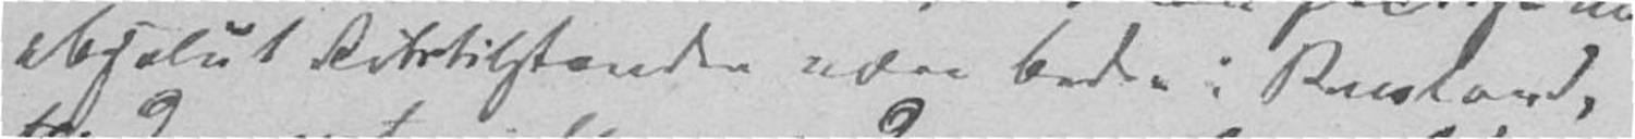absolut Retstilstanden være bedre i Rusland,
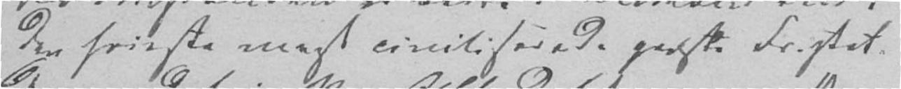den frieste mest civiliserede græske Fristat.
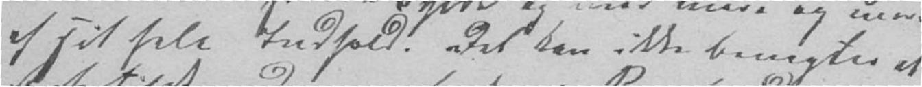af sit hele Indhold. Det kan ikke benægtes at
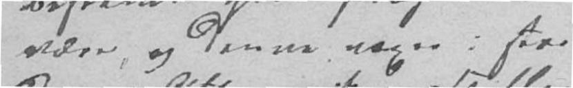være, og denne voxer i stor
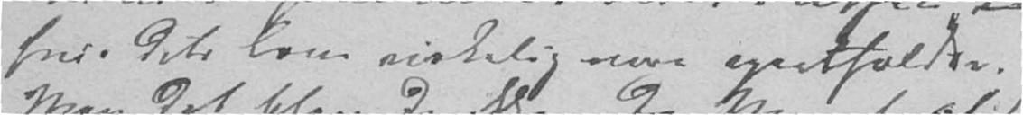hvis dets Love virkelig vare opretholdte.
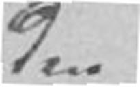den
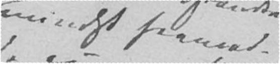mindst fremad-
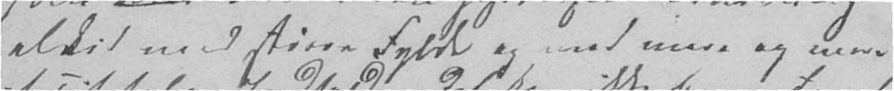altid med større Fylde og med mere og mere
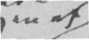en af
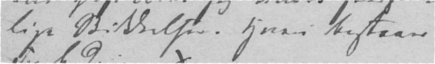lige Skikkelser. Hvori bestaaer
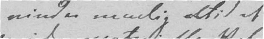vinder nemlig altid et
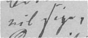vil sige,
